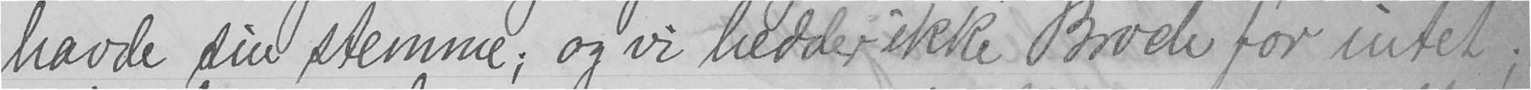havde sin stemme; og vi hedder ikke Broch for intet;
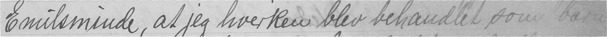Emilsminde, at jeg hverken blev behandlet som barn
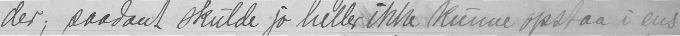der; saadant skulde jo heller ikke kunne opstaa i ens
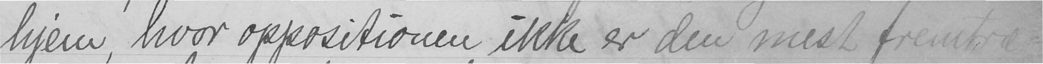hjem, hvor oppositionen ikke er den mest fremtræ-
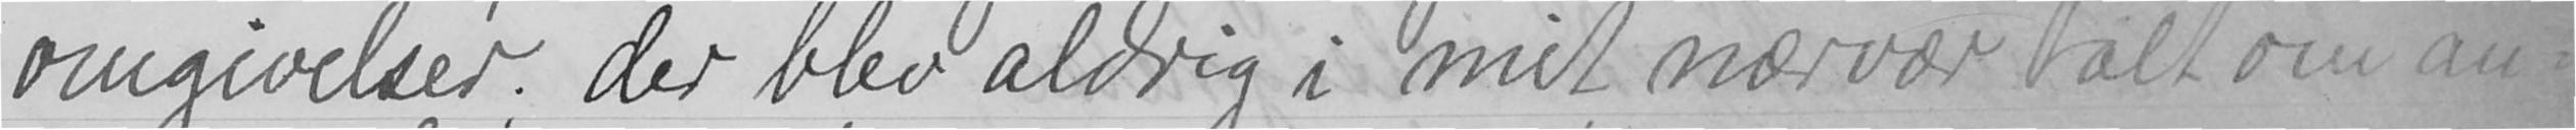omgivelser; der blev aldrig i mit nærvær talt om an-
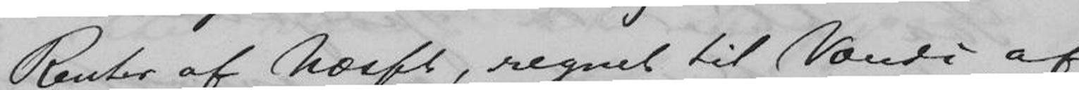Renter af Næsset, regnet til Værdi af
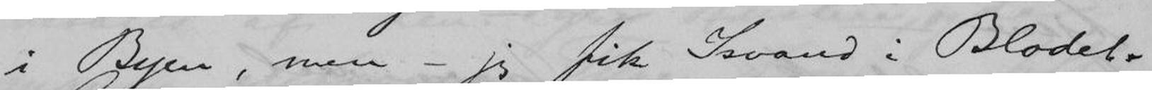i Byen, men - jeg fik Isvand i Blodet.
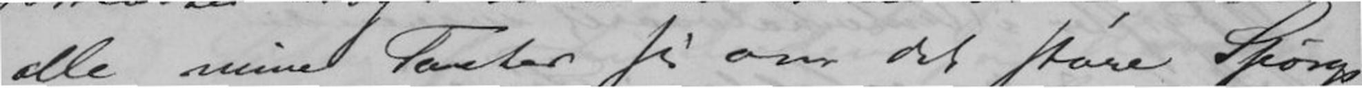alle mine Tanker sig om det store Spørgs-
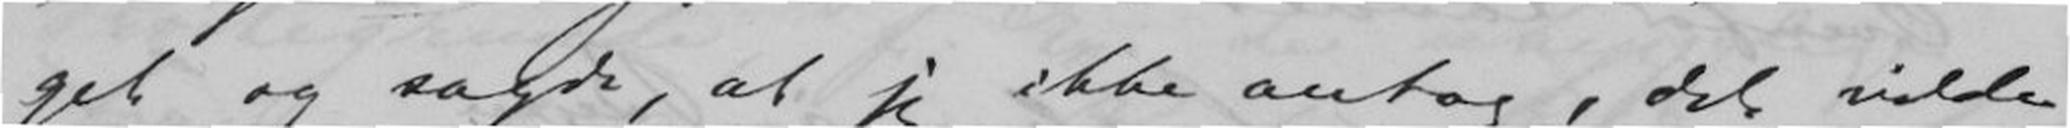get og sagde, at jeg ikke antog, det vilde
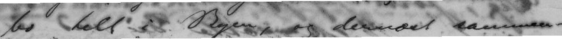bo helt i Byen, og dernæst sammen-
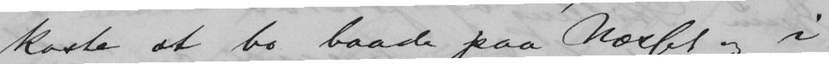koste at bo baade paa Næsset og i
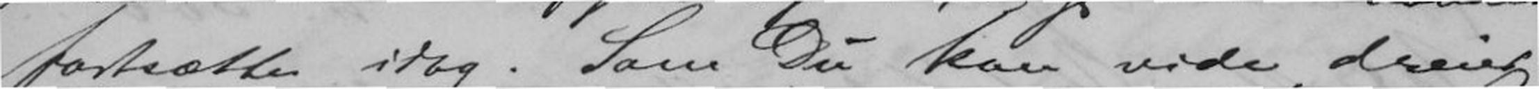fortsætte idag. Som Du kan vide, dreier
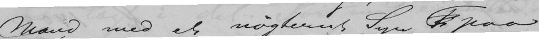Mand med et nøgternt Syn paa
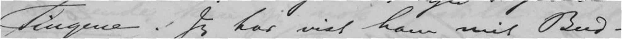Tingene. Jeg har vist ham mit Bud-
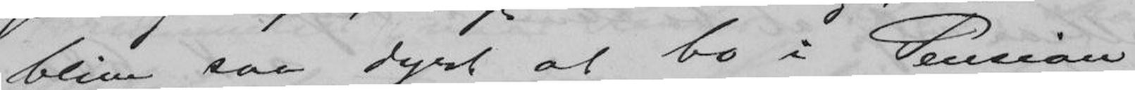blive saa dyrt at bo i Pension
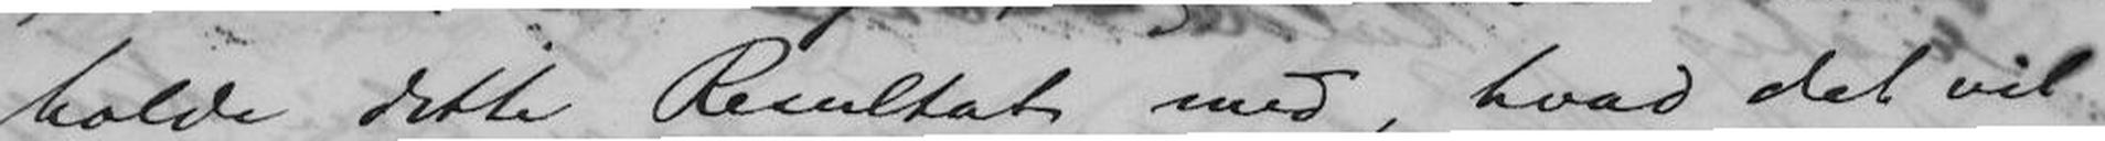holde dette Resultat med, hvad det vil
